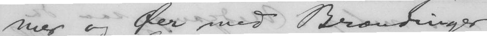mer og Øer med Brændinger
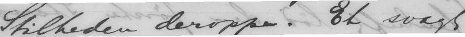Stilheden deroppe. Et svagt
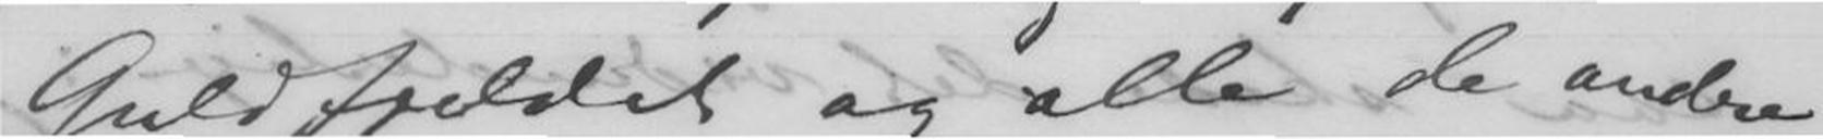Guldfjeldet og alle de andre
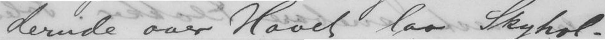derude over Havet laa Skyhol-
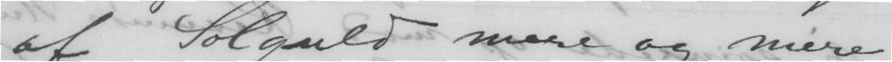af Solguld mere og mere
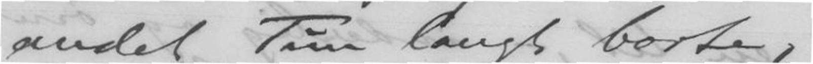andet Tun langt borte,
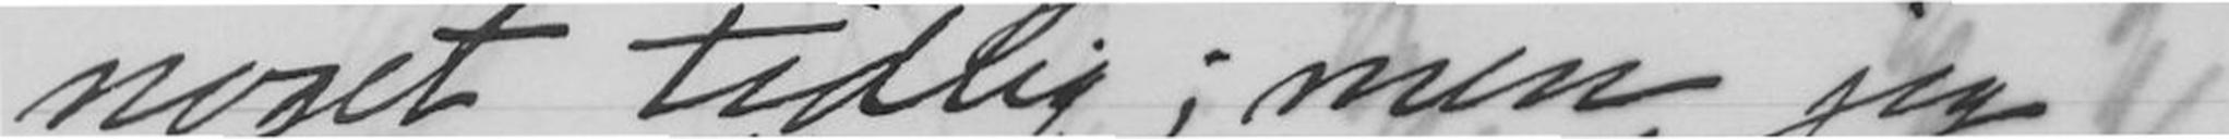noget tidlig; men jeg
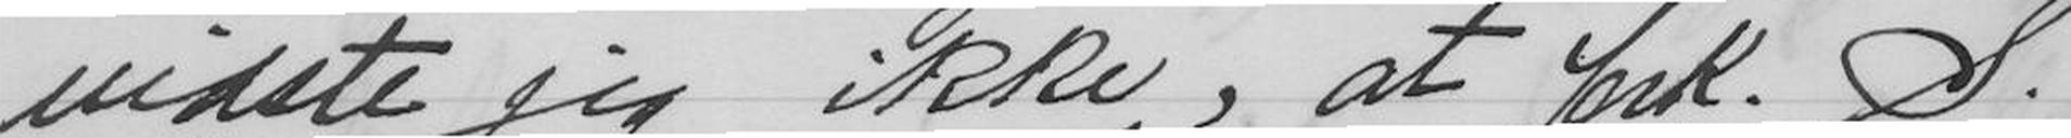vidste jeg ikke, at frk. S.
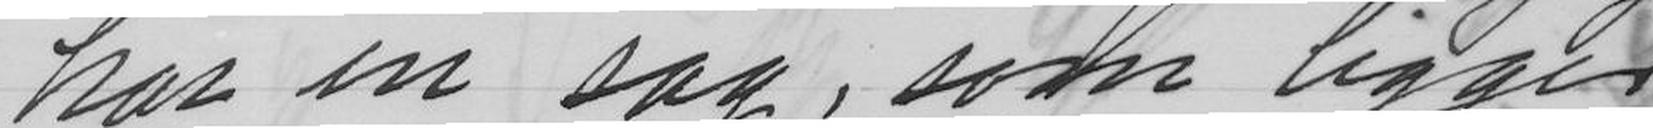har en sag, som ligger
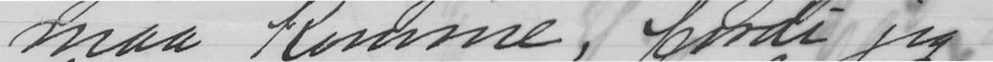maa komme, fordi jeg
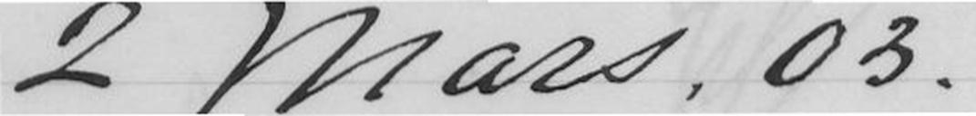2 Mars. 03.
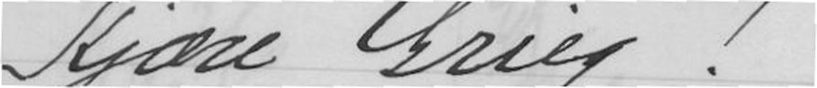Kjære Grieg!
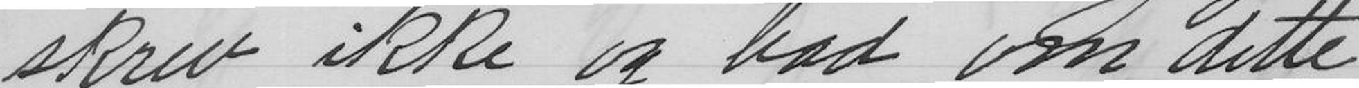skrev ikke og bad om dette,
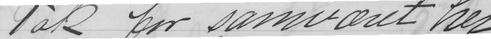Tak for samværet her,
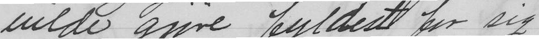vilde gjøre fyldest for sig
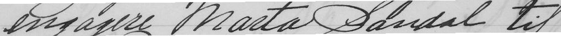engagere Marta Sandal til
